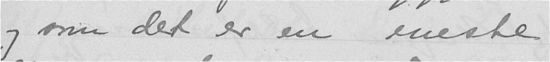og som det er en eneste
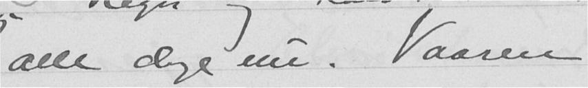alle dage nu. Vaaren
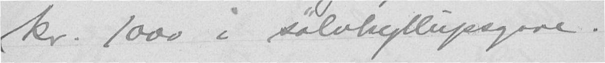kr. 1000 i sølvbryllupsgave.
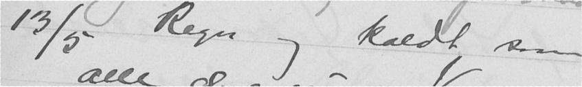13/5 Regn og kaldt som
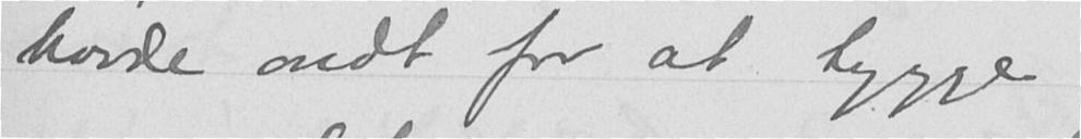havde ondt for at tygge
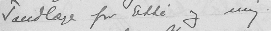Tandlæge for Etti og mig.
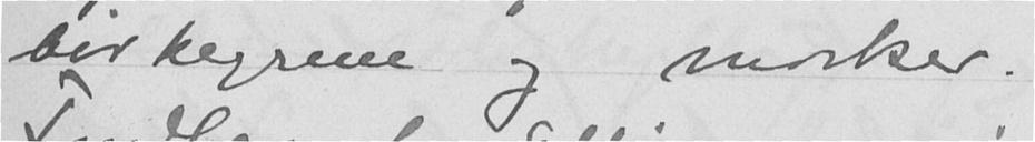birkegrene og marker.
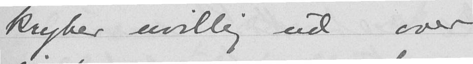kryber uvillig ud over
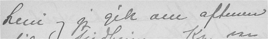Leni og jeg gik om aftenen
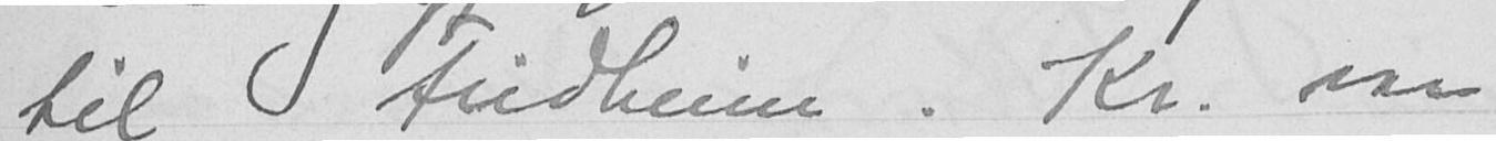til Fridheim. Kr. var
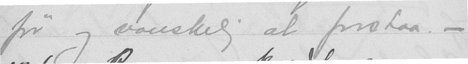før og vanskelig at forstaa. -
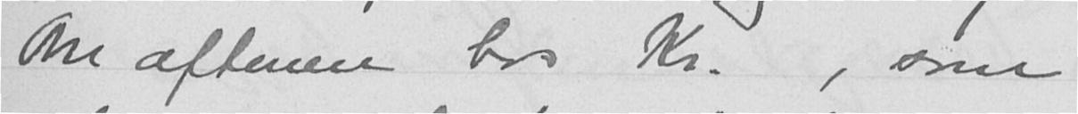Om aftenen hos Kr., som
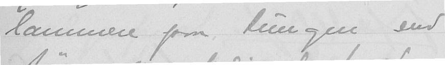lammere paa lungen end
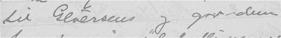til Gløersens og gav dem
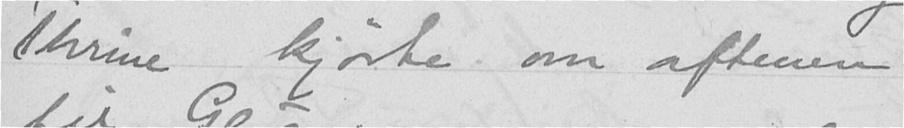Thrine kjørte om aftenen
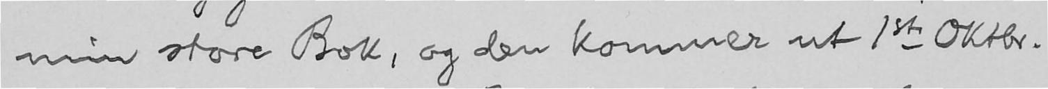min store Bok, og den kommer ut 1ste Oktbr.
